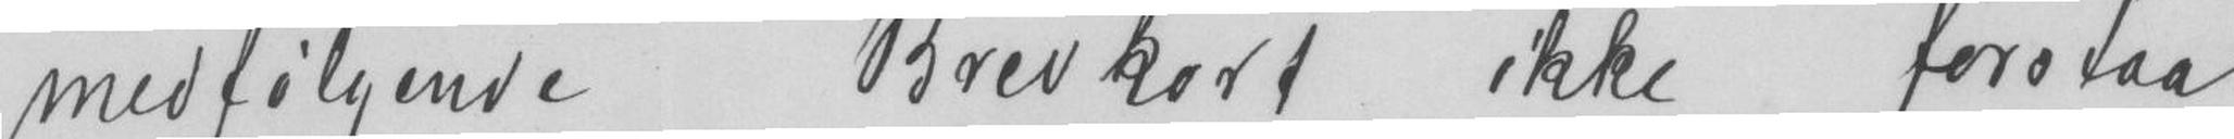medfølgende Brevkort ikke forstaa
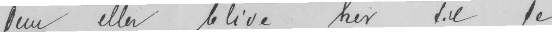Dem eller blive her til De
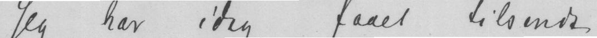Jeg har idag faaet tilsendt
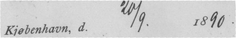Kjøbenhavn. d. 20/9 1890
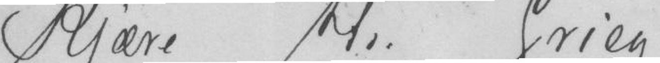Kjære Hr. Grieg!
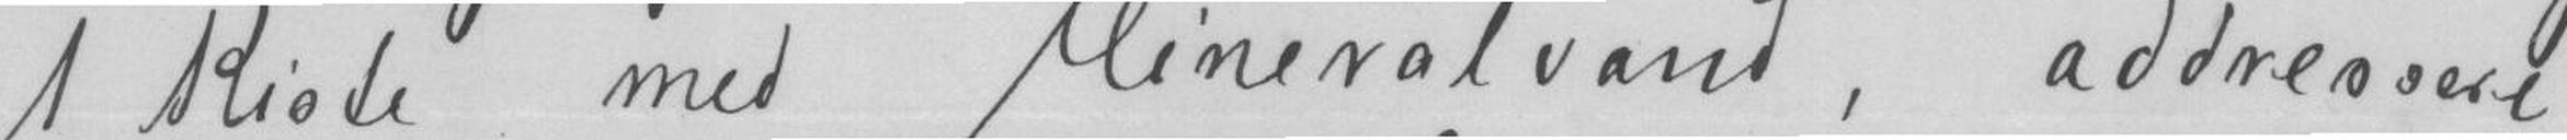1 kiste med Mineralvand, addressere
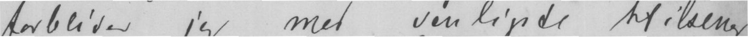Forbliver jeg med venligste Hilsener
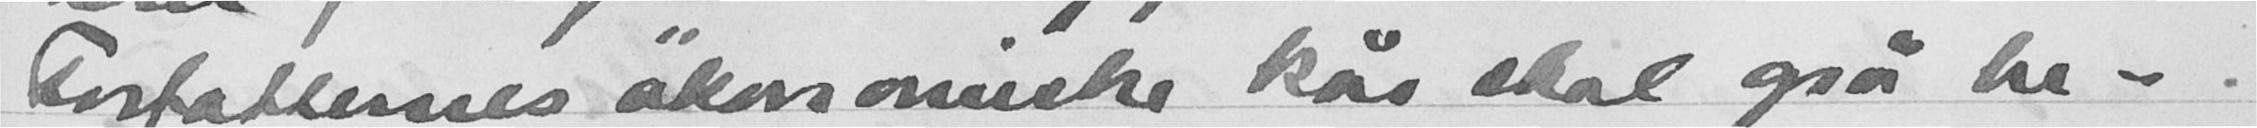Forfatternes økonomiske kår skal også be-
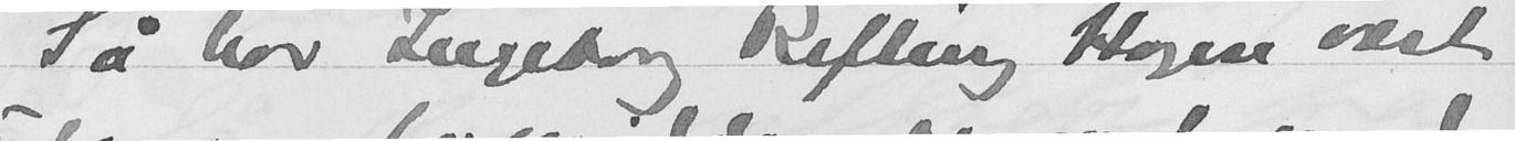Så har Ingeborg Refling Hagen vært
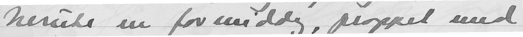herute en formiddag, proppet med
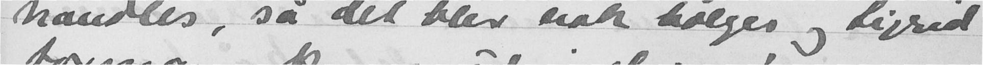handles, så det blir nok bølger og Sigrid,
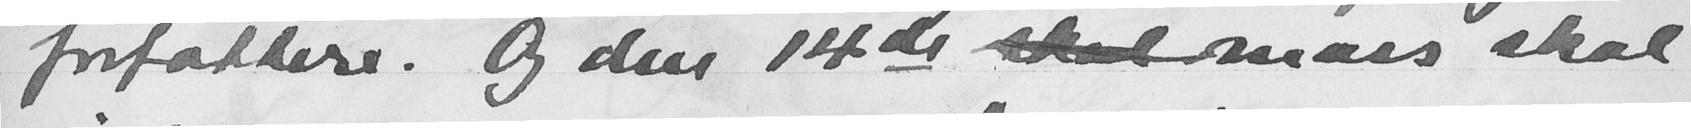forfattere. Og den 14de  mars skal
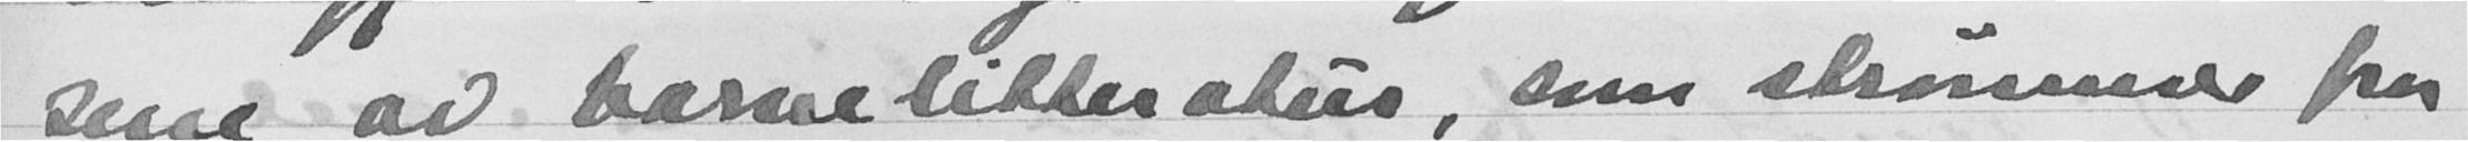sene av barnelitteratur, som strømmer fra
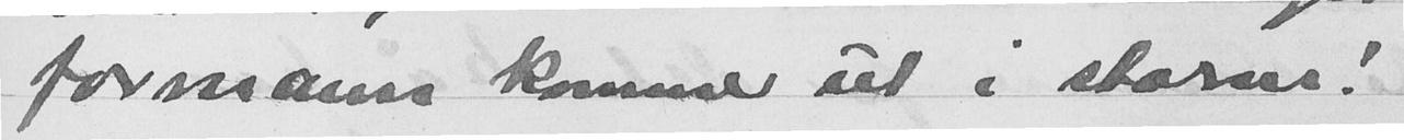formann kommer ut i storm!
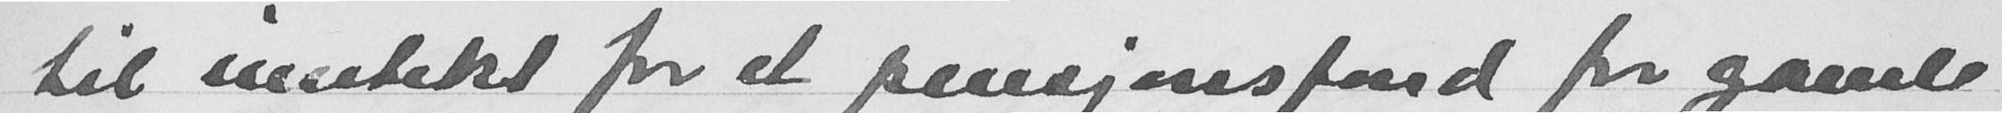til inntekt for et pensjonsfond for gamle
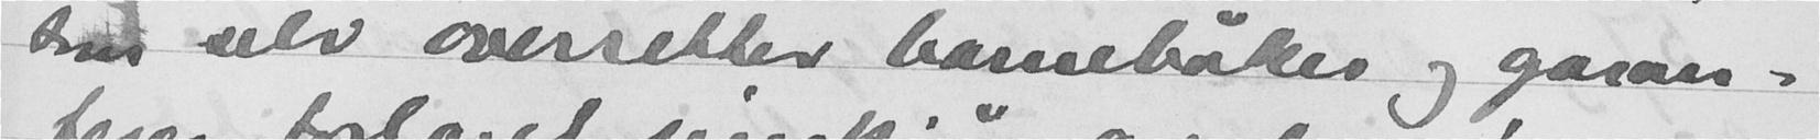som selv oversetter barnebøker og garan-
